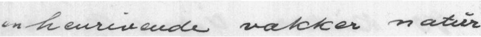en henrivende vakker natur
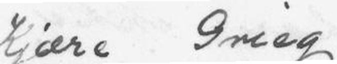Kjære Grieg!
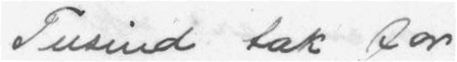Tusind tak for
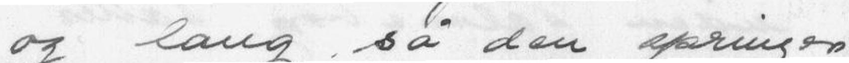og lang, så den springer
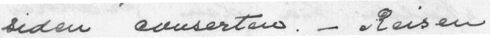siden concerten. - Reisen
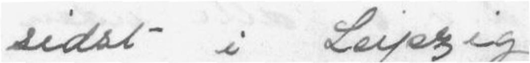sidst i Leipzig! -
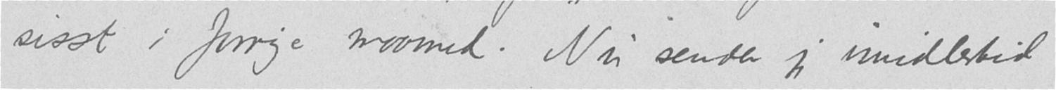sisst i forrige maaned. Nu sender jeg imidlertid
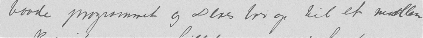baade programmet og Deres brev op til et medlem
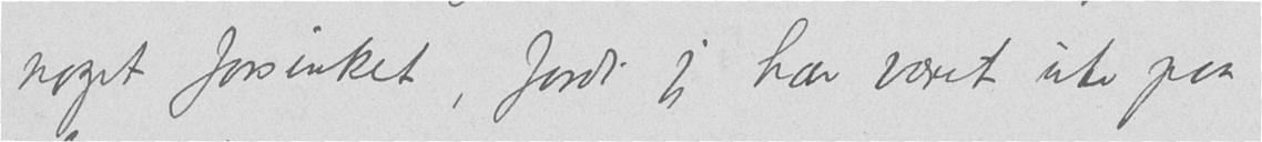noget forsinket, fordi jeg har været ute paa
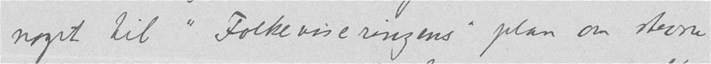noget til "Folkeviseringens" plan om stevne
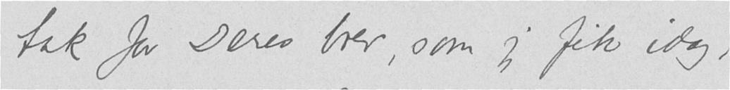tak for Deres brev som jeg fik idag,
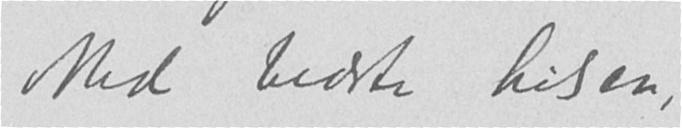Med bedste hilsen,
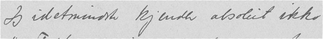Jeg idetmindste kjender absolut ikke
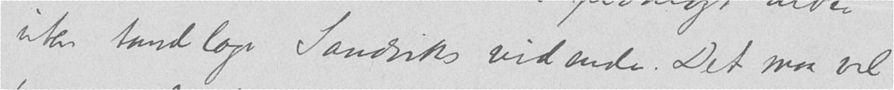uten tandlæge Sandviks vidende. Det maa vel
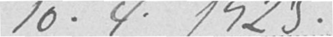10.4. 1923
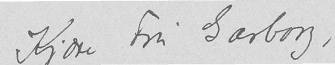Kjære Fru Garborg,
